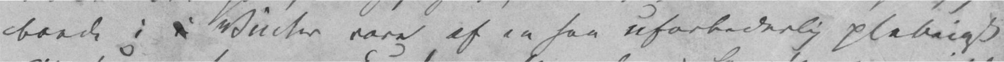boede i i Vinter vare af en saa uforbederlig plebeisk
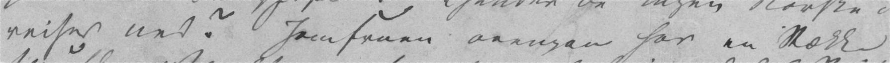reiser ned? Jomfruen ovenpaa har en Række
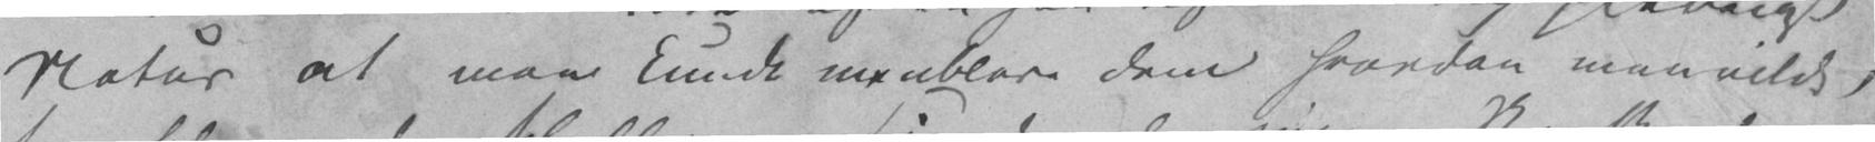Natur at man kunde meublere dem hvordan man vilde,
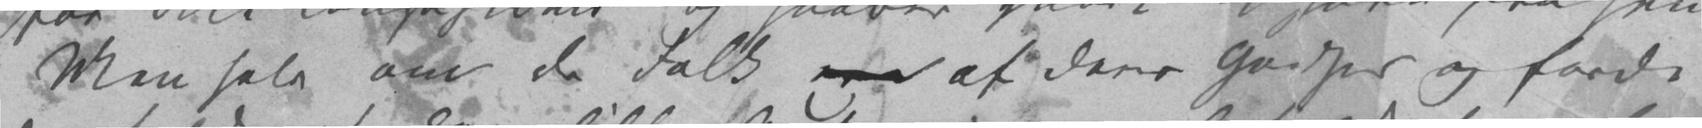Men selv om de Folk ere af deres Godhed og fordi
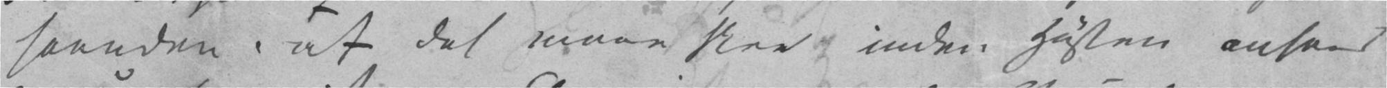Haanden, at det maae skee inden Høsten anseer
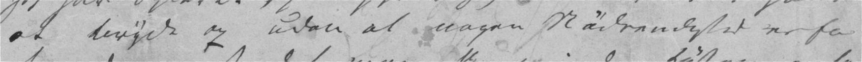at bryde op uden at nogen Nødvendighed er for
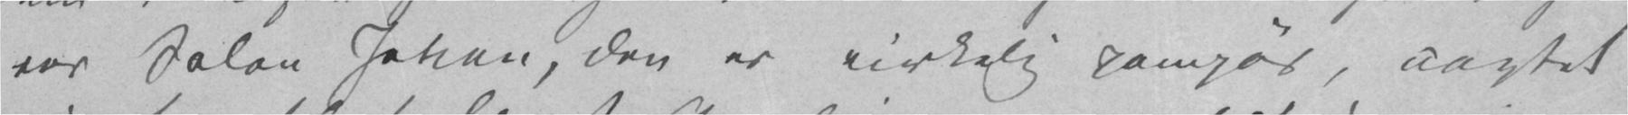vor Salon Johan, den er virkelig pompøs, uagtet
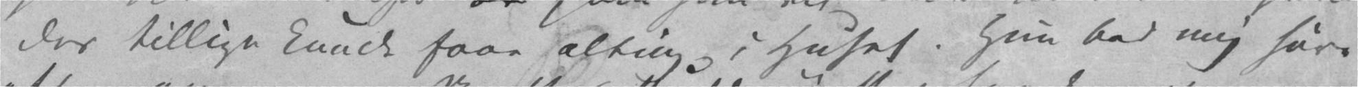der tillige kunde faae alting i Huset. Hun bad mig høre
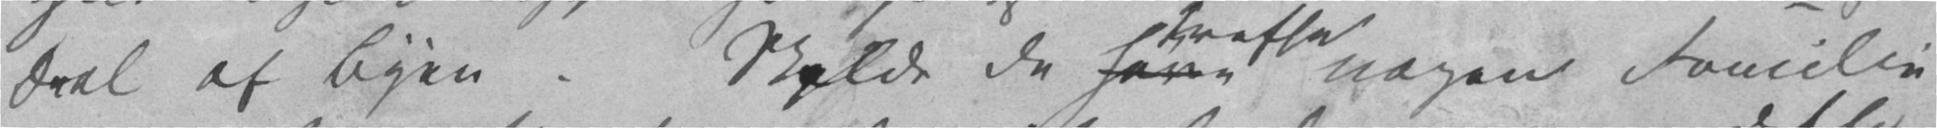Deel af Byen. Skulde De have nogen Familie
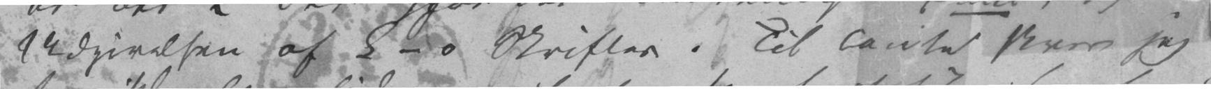Udgivelsen af C-s Skrifter. Til Tante skrev jeg
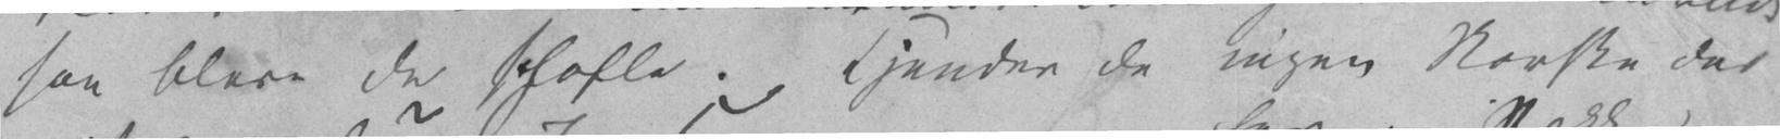saa bleve de schofle. Kjender De ingen Norske der
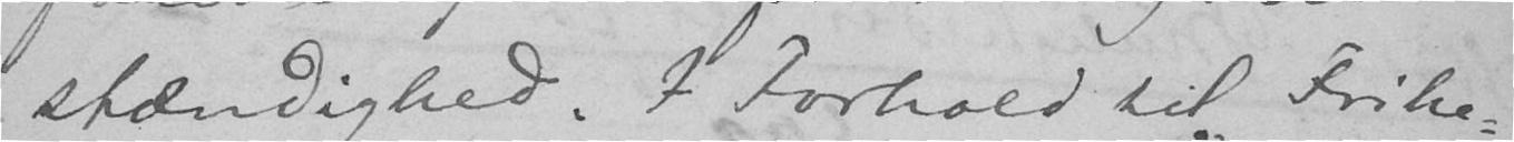stændighed. I Forhold til Frihe-
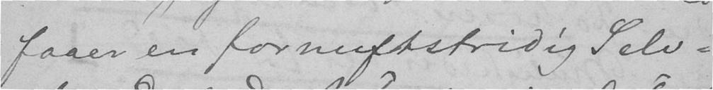faaer en fornuftstridig Selv-
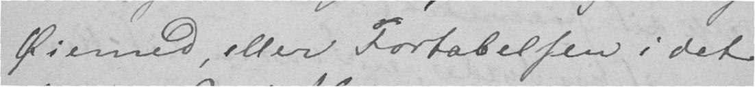Øiemed, eller Fortabelsen i det
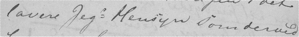lavere Jegs Hensyn som derved
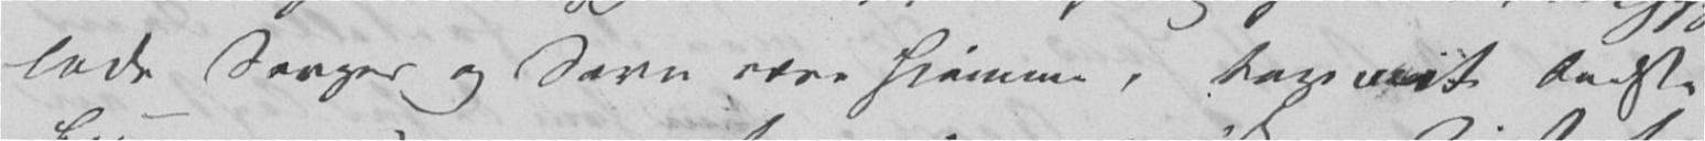lade Sorger og Savn være hjemme, tage mit bedste
